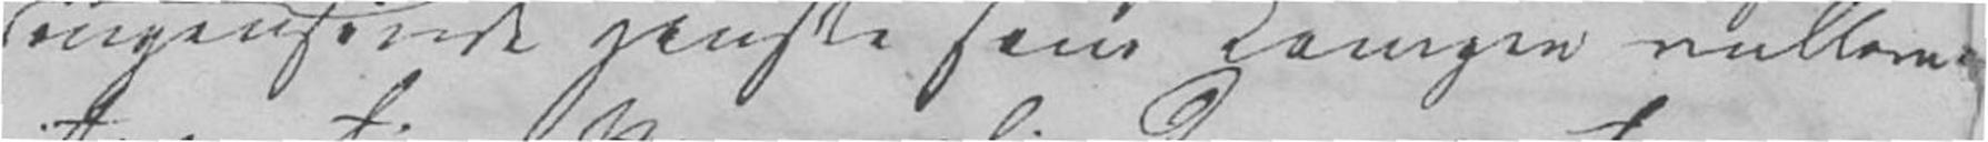ingensinde ganske som Kampen mellem
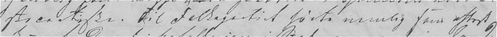stocratiske. Til Folkepartiet hørte nemlig som oftest
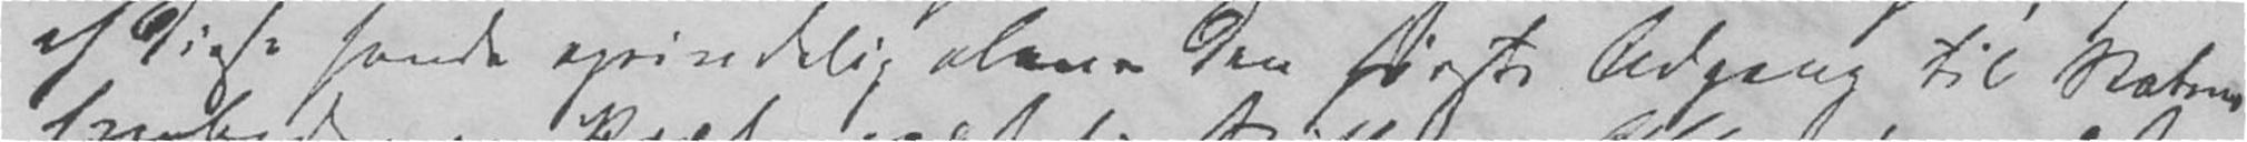af disse havde oprindelig alene den første Adgang til Statens
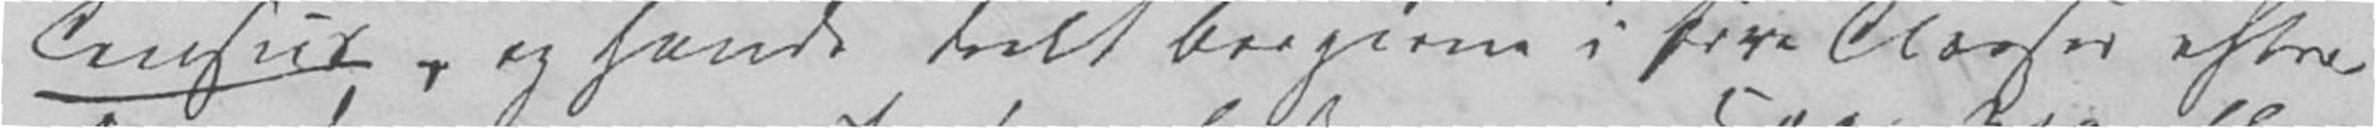Census, og havde deelt Borgerne i fire Classer efter-
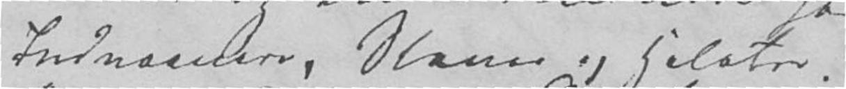Indvaanere, Slaver og Heloter.
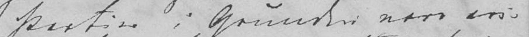Partier i Grunden vare ari-
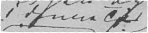i denne Tid
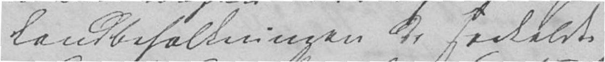Landbefolkningen de saakaldte
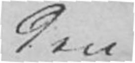den
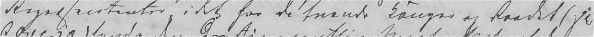Repræsentanter i det hos de tvende Konger og Raadet (X
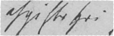afgiftsfri
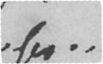Bor-
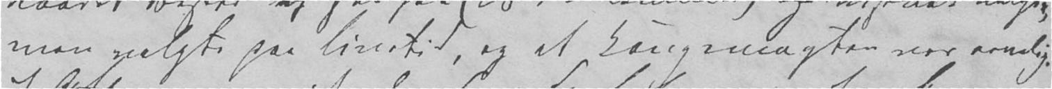men valgte paa Livstid, og at Kongemagten var arvelig.
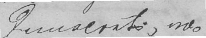Democrati, væ-
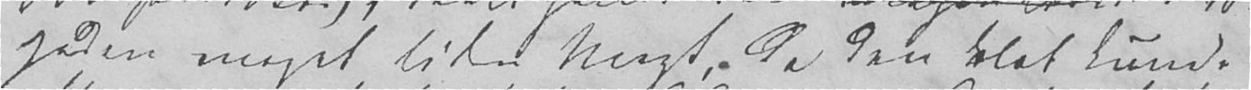heden meget liden Magt, da den blot kunde
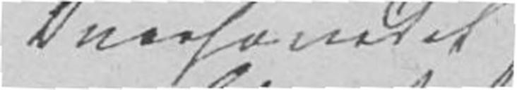Overhovedet
